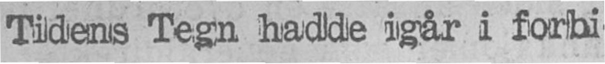Tidens Tegn hadde igår i forbi-
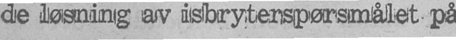de løsning av isbryterspårsmålet på
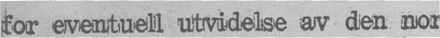for eventuell utvidelse av den nor-
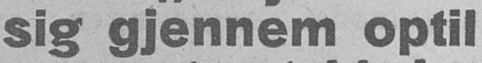sig gjennem optil
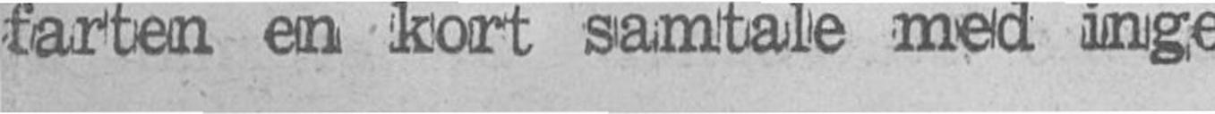farten en kort samtale med inge-
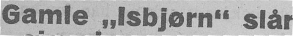Gamle „Isbjørn“ slår
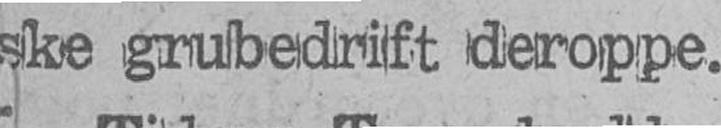ske grubedrift deroppe.
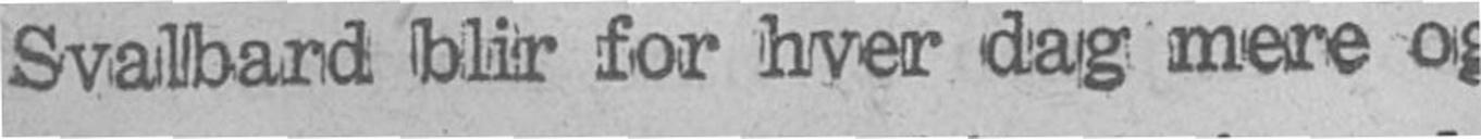Svalbard blir for hver dag mere og
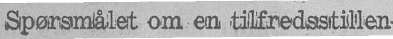Spørsmålet om en tilfredsstillen-
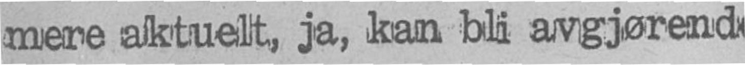mere aktuelt, ja, kan bli avgjørende
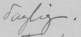daglig.
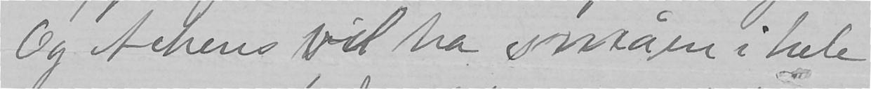Og Achens vil ha småen i hele
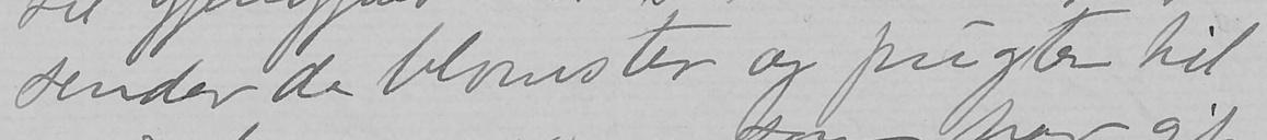sender de blomster og frugter til
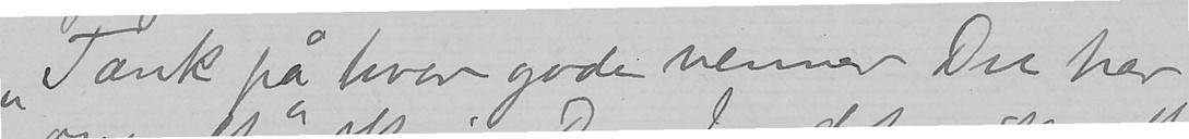"Tænk på hvor gode venner Du har
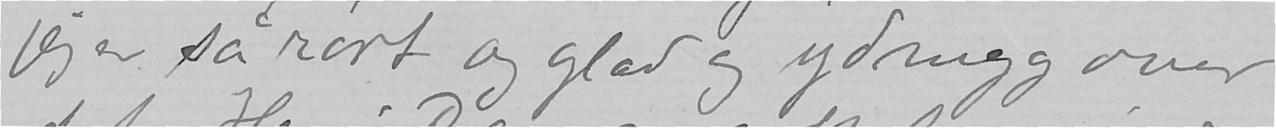jeg er så rørt og glad og ydmyg over
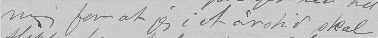mig for at jeg i et årstid skal
